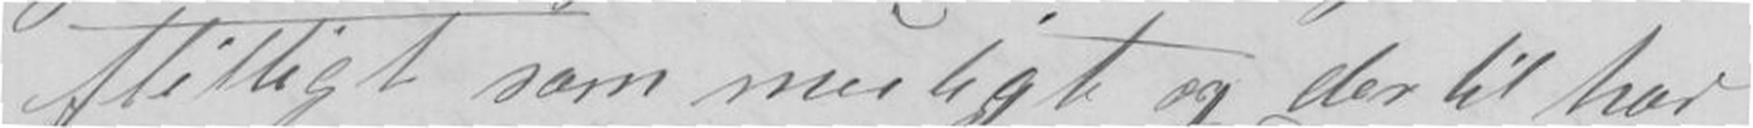flittigt som muligt og der til har
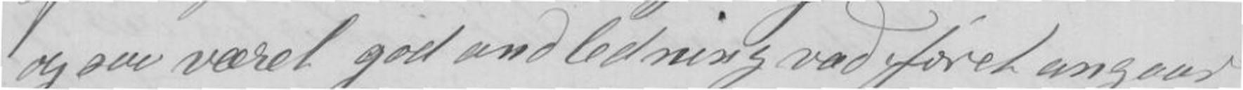ogsaa været god andledning vad føret angaar
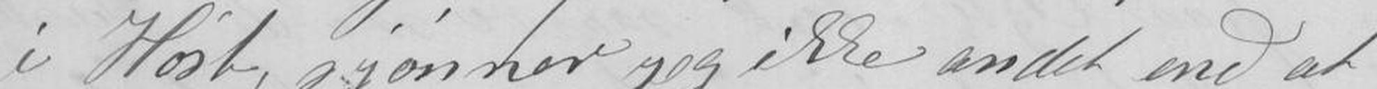i Høst, sjønner jeg ikke andet end at
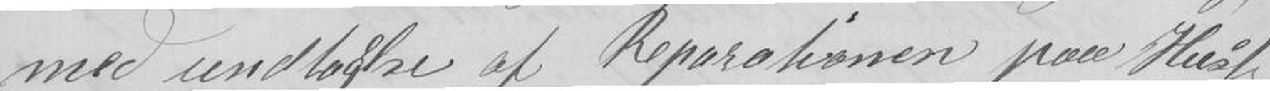med undtagelse af Reparationer paa Huset
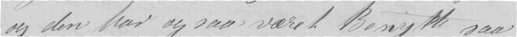og den har ogsaa været Benytte saa
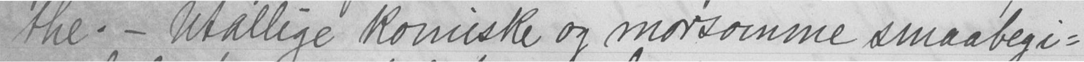the. - Utallige komiske og morsomme smaabegi-
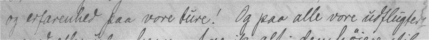og erfarenhed paa vore ture! Og paa alle vore udflugter
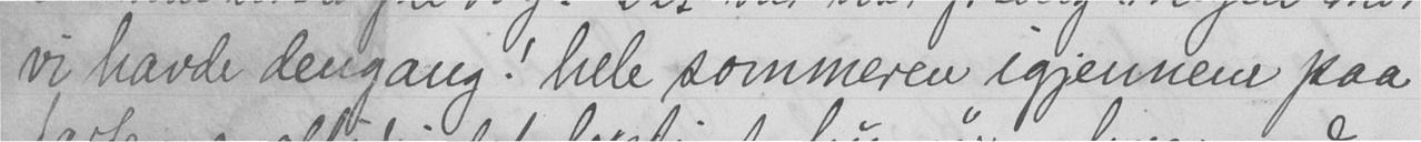vi havde dengang! hele sommeren igjennem paa
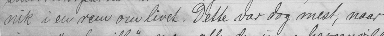nik i en rem om livet. Dette var dog mest, naar
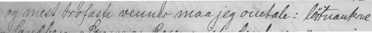og mest trofaste venner maa jeg omtale: løitnanterne
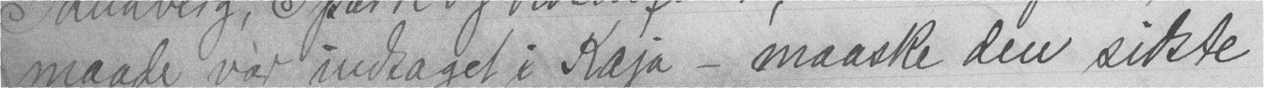maade var indtaget i Kaja - maaske den sidste
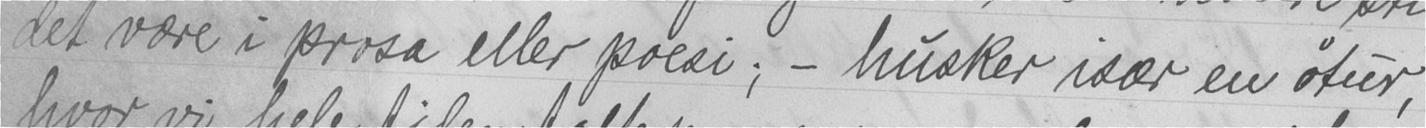det være i prosa eller poesi; - husker især en øtur,
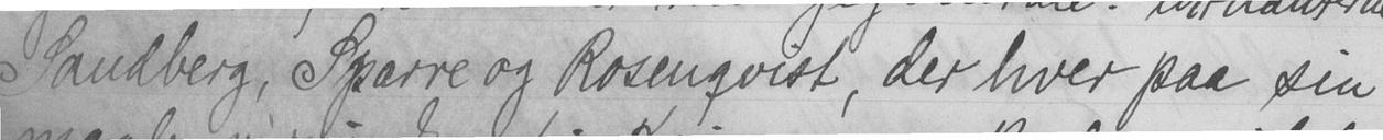Sandberg, Sparre og Rosenqvist, der hver paa sin
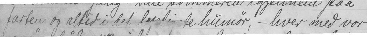farten og altid i det lepligste humør, - hver med vor
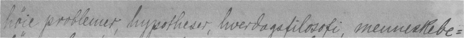høie problemer, hypotheser, hverdagsfilosofi, menneskebe-
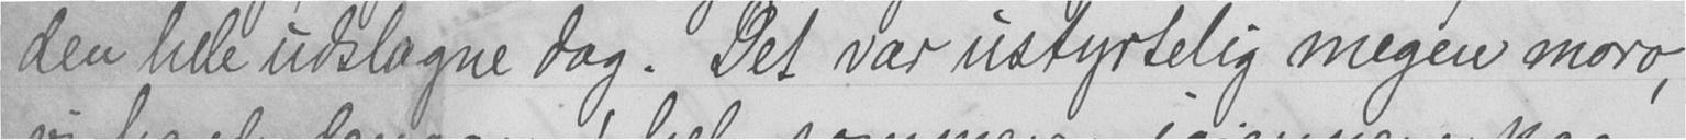den hele udslagne dag. Det var ustyrtelig megen moro,
